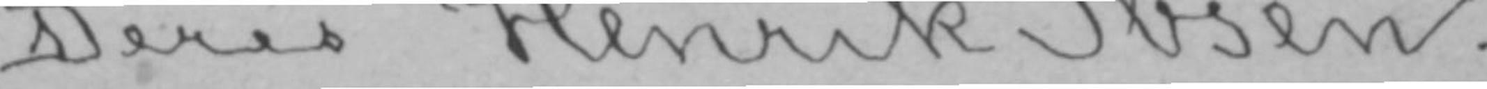Deres Henrik Ibsen.
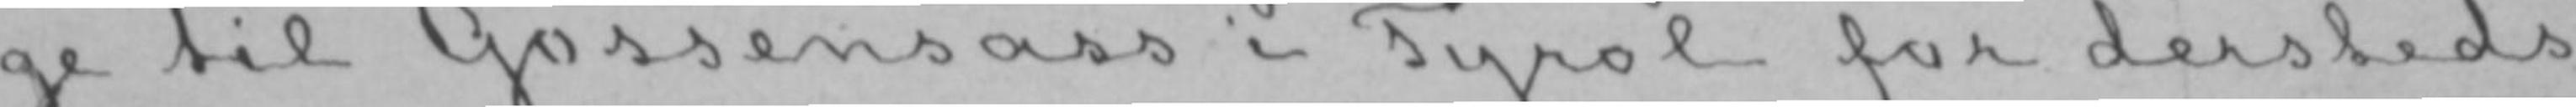ge til Gossensass i Tyrol for dersteds
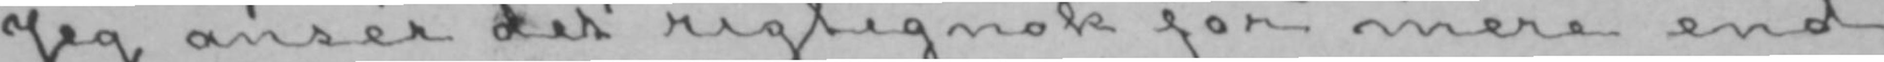Jeg ansér det rigtignok for mere end
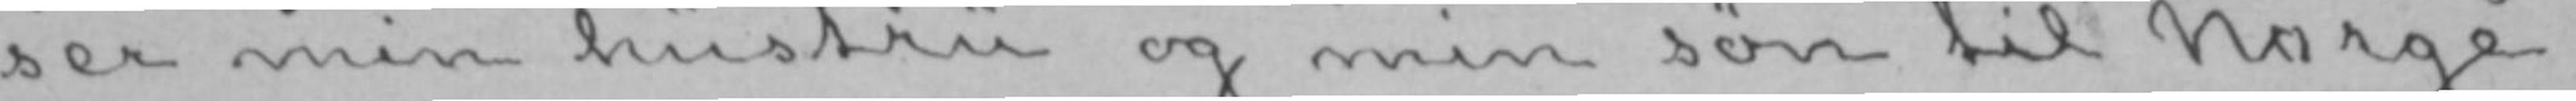ser min hustru og min søn til Norge.
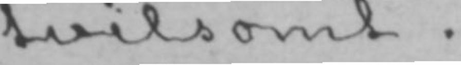tvilsomt.
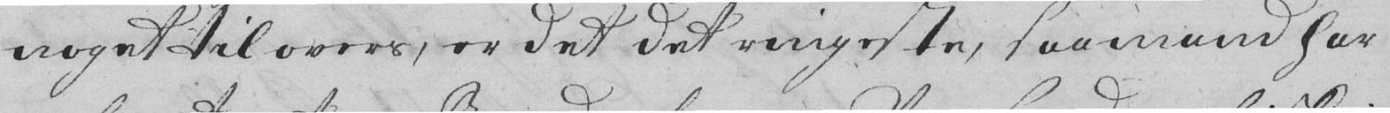noget til overs, er det det ringeste, saa mand har
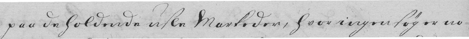paa de holdende usle Markeder, hvor ingen søger no-
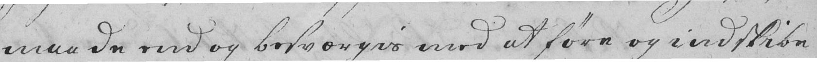maa de end og besværgis med at føre og indskibe
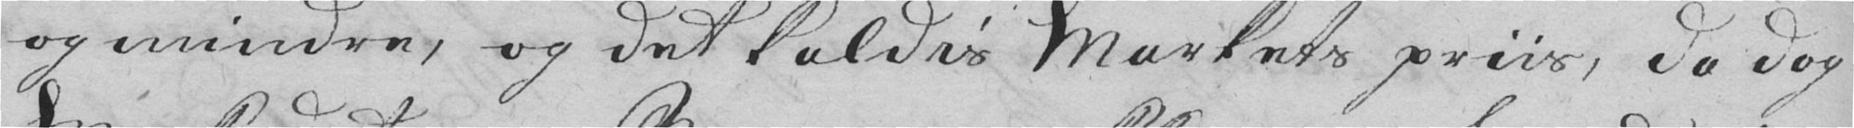og mindre, og det kaldes Markets priis, da dog
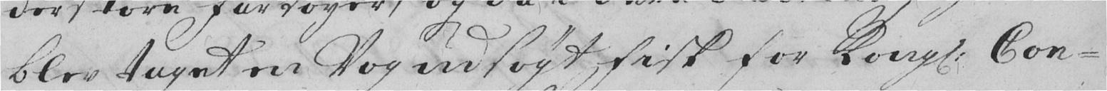blev taget en Vog udsøgt Fisk for Kongl. Con-
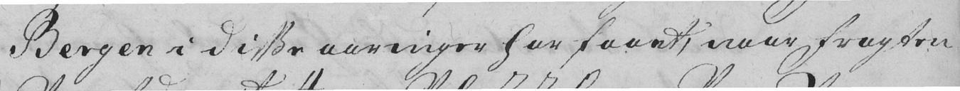Bergen i disse aaringer har faaet, naar Fragten
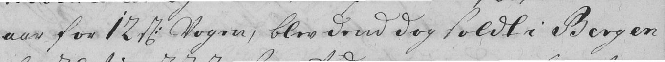aar for 12 sk. Vogen, blev dend dog soldt i Bergen
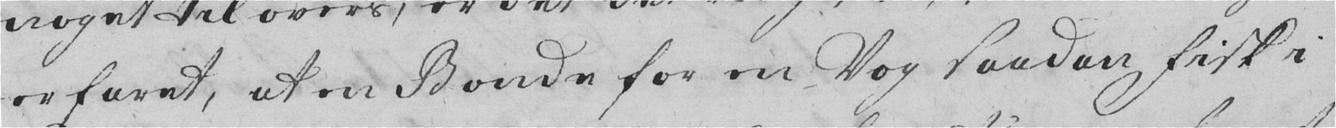erfaret, at en Bonde for en Vog saadan Fisk i
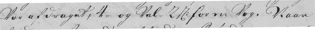Var afdraget, 4 og Vel 2 sk. for en Vog. Naar
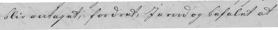blir antaget, fordret, Ia end og befalet at
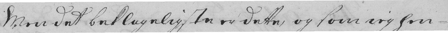Men det beklageligste er dette, og som ieg hen-
